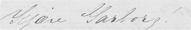Kjære Garborg!
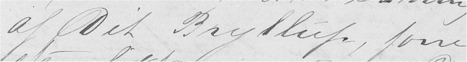af Dit Bryllup, forre-
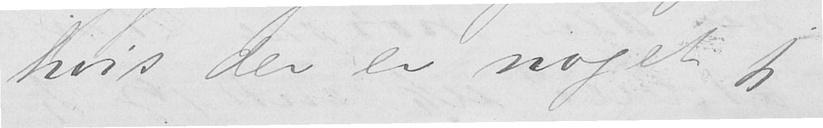hvis der er noget jeg
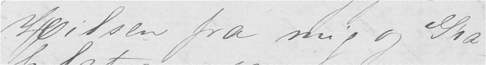Hilsen fra mig og Gra-
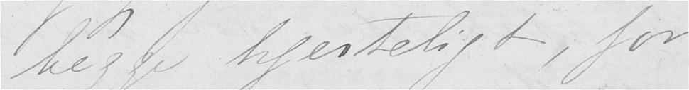begge hjerteligt, for
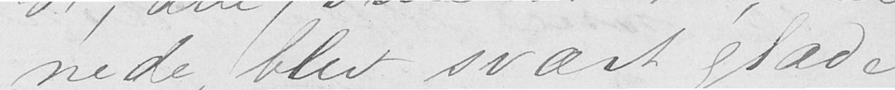nede, blev svært glade
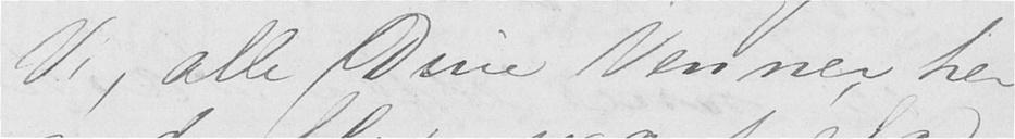Vi, alle Dine Venner her
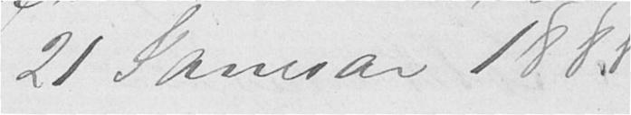21 Januar 1888
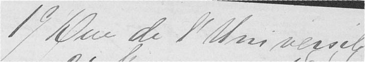19 Rue de l'Universit
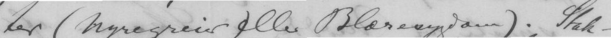ter (Nyregreier overskrevet: ) eller Blæresygdom). Stak-
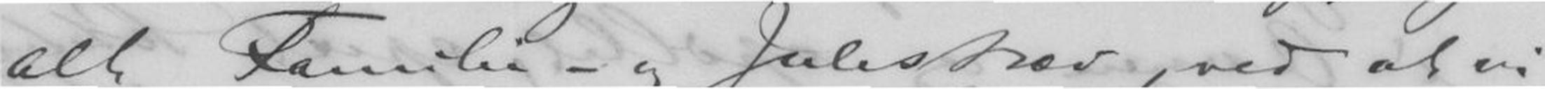alt Familie- og Julestræv, ved at vi
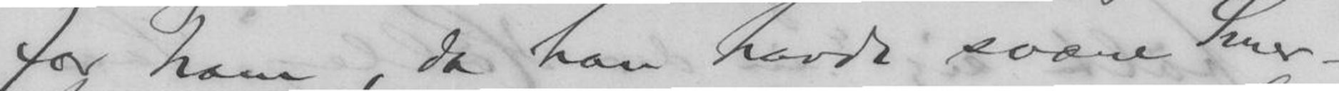for ham, da han havde svære Smer-
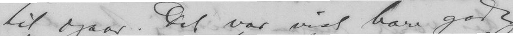til igaar. Det var vist bare godt
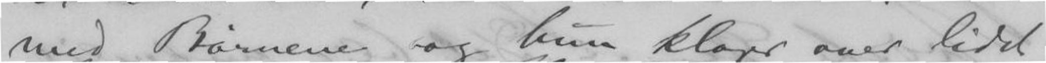med Børnene og hun klager over lidet
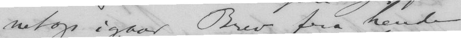netop igaar Brev fra hende,
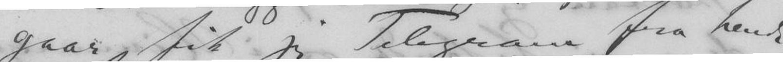i gaar fik jeg Telegram fra hende,
